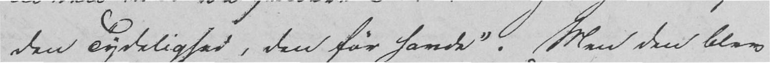den Tydelighed, den før havde". Men den blev
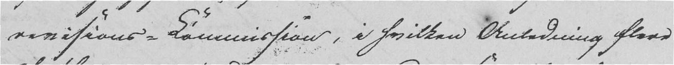revisions-Kommission, i hvilken Anledning flere
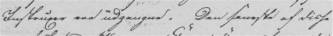Instruxer ere udgangne. Den seneste af disse
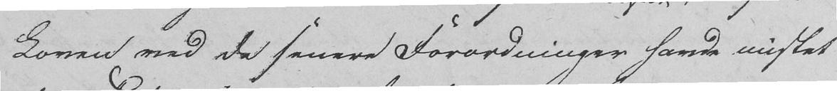Loven ved de senere Forordninger havde mistet
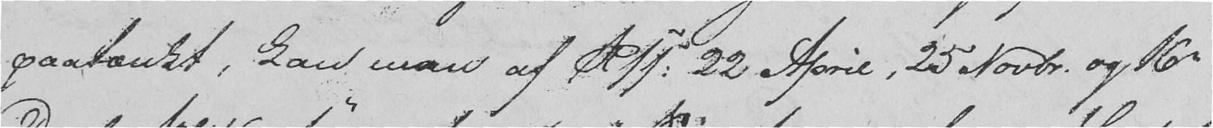paatænkt, kan man af Res. 22 April, 25 Novbr. og 16
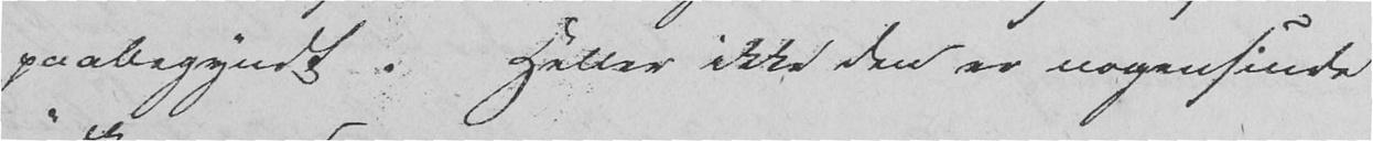paabegyndt. Heller ikke den er nogensinde
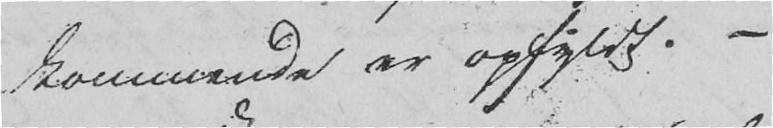kommende er opfyldt. -
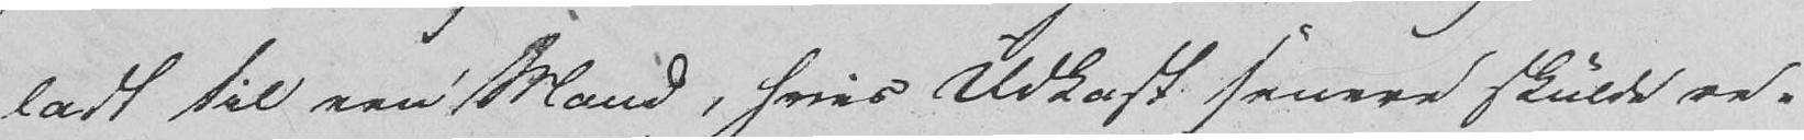ladt til een Mand, hvis Udkast senere skulde re-
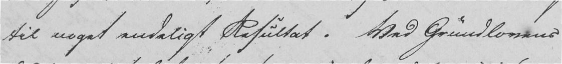til noget endeligt Resultat. Ved Grundlovens
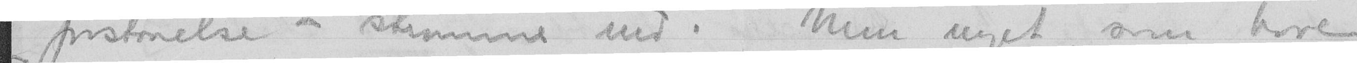"forstaaelse" strømme ind. Men noget, som bare
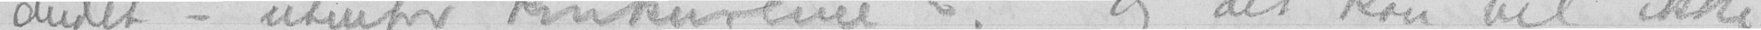andet - utenfor konkurence! Og det kan vel ikke
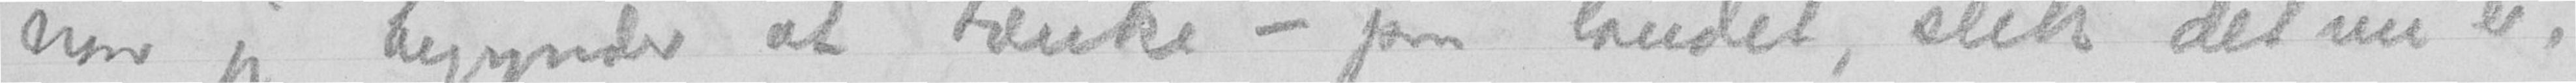naar jeg begynder at tænke - paa landet, slik det nu er,
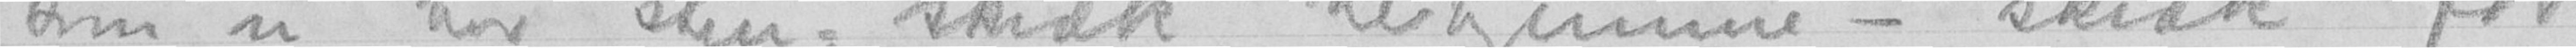som vi har sten-skræk, herhjemme - skræk for
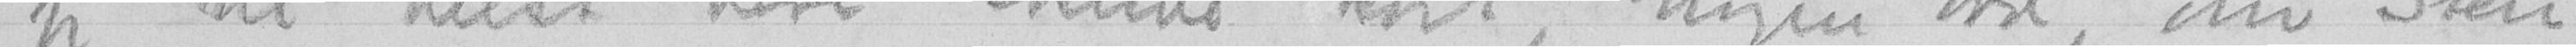jeg vil helst bare skrive kort, nogen ord, om sten
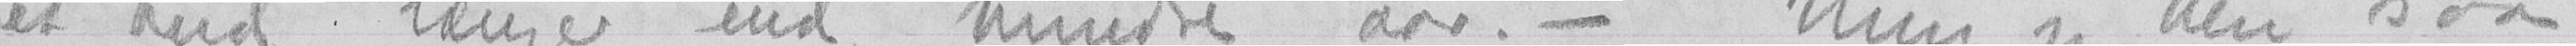et bud længer end hundre aar. - Men jeg blir saa
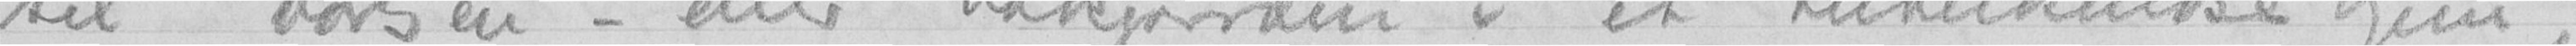til børsen - eller bakgaarden i et tuberkulosehjem,
